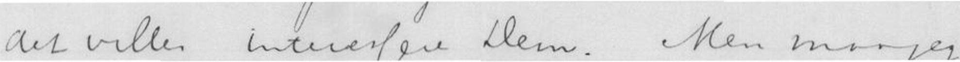det ville interessere Dem. Men maa jeg
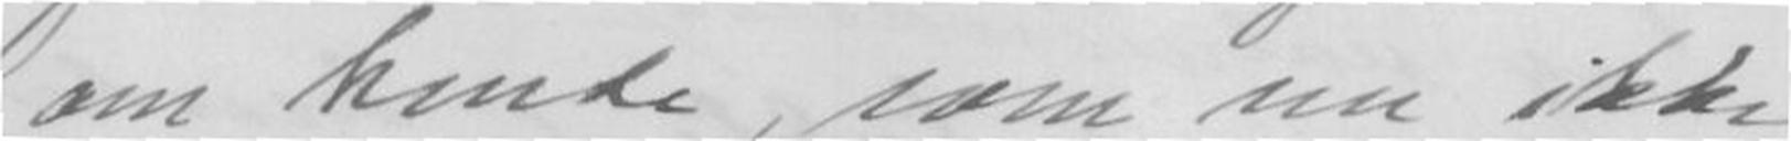om hende, som nu ikke
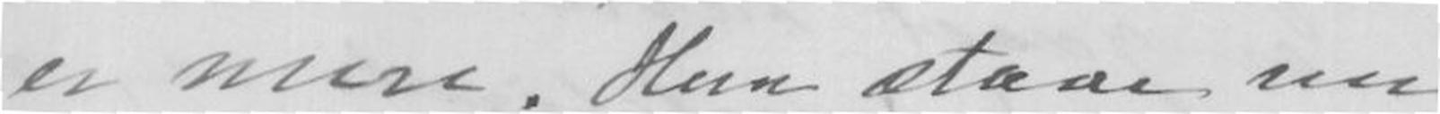er mere. Hun staar nu
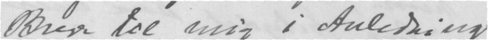Brev til mig i Anledning
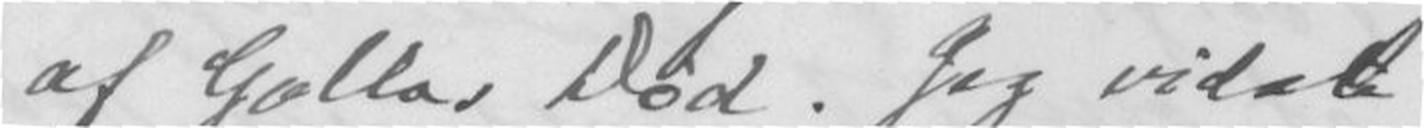af Gollas Død. Jeg vidste
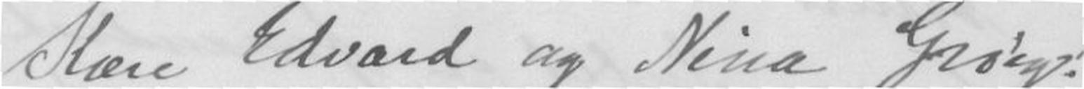Kære Edvard og Nina Grieg!
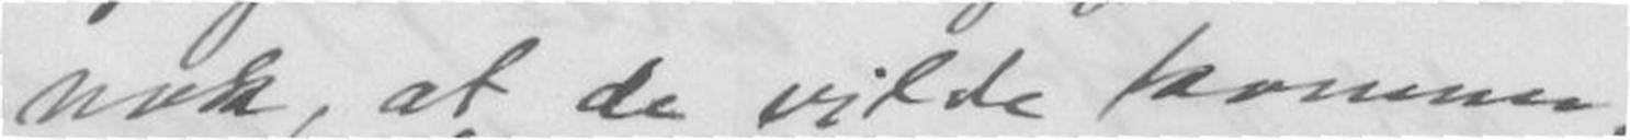nok, at de vilde komme,
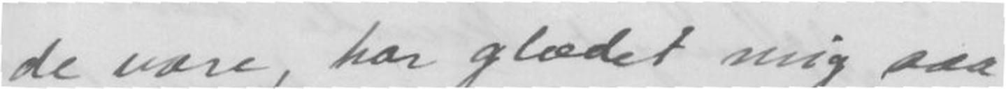de være, har glædet mig saa
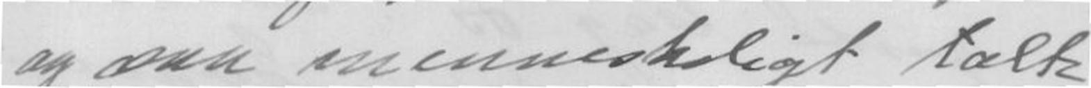og saa menneskeligt talte
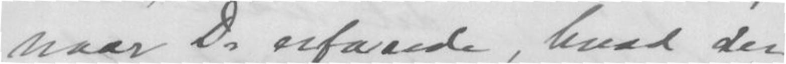naar De erfarede, hvad der
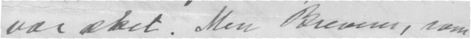var sket. Men Brevene, som
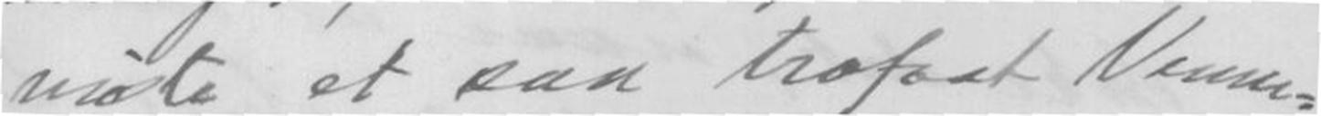viste et saa trofast Venne-
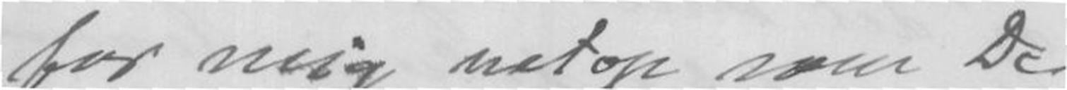for mig netop som De
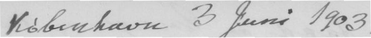København 3 Juni 1903
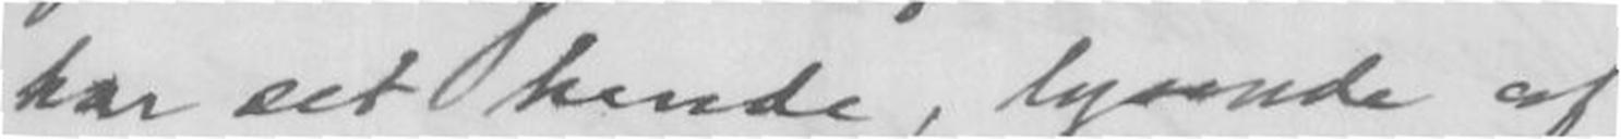har set hende, lysende af
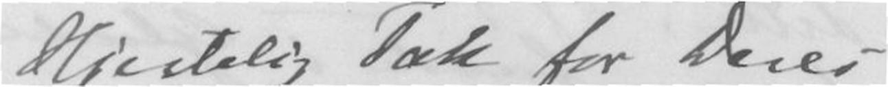Hjertelig Tak for Deres
